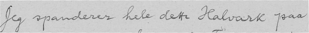Jeg spanderer hele dette Halvark paa
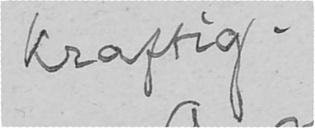kraftig.
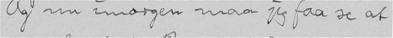Og nu imorgen maa jeg faa se at
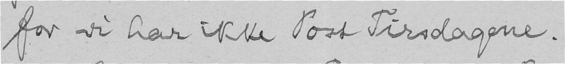for vi har ikke Post Tirsdagene.
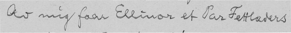Av mig faar Ellinor et Par Fettlæders
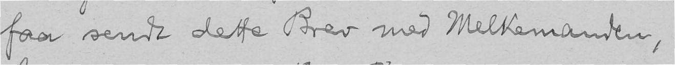faa sendt dette Brev med Melkemanden,
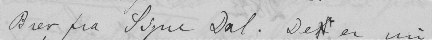Brev fra Signe Dal. De er nu
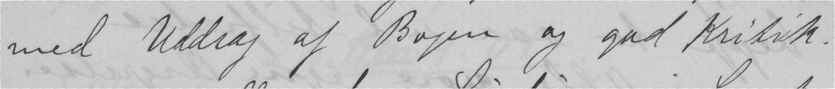med Uddrag af Bogen og god Kritik.
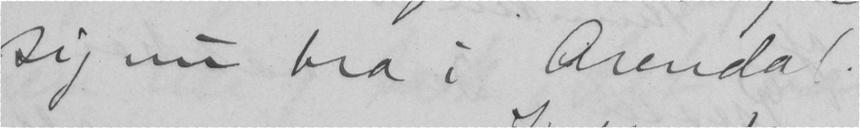sig nu bra i Arendal.
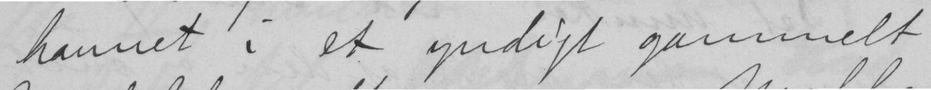havnet i et yndigt gammelt
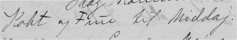Koht og Frue til Middag.
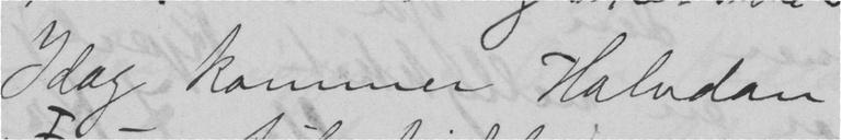Idag kommer Halvdan
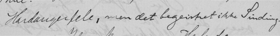Hardangerfele, men det begeistret ikke Sinding.
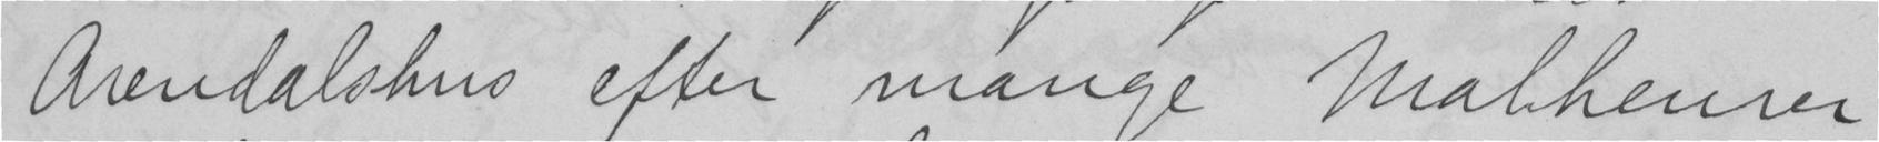Arendalshus efter mange malheurer
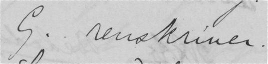G. renskriver.
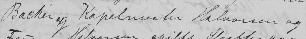Backer og Kapelmester Halvorsen og
